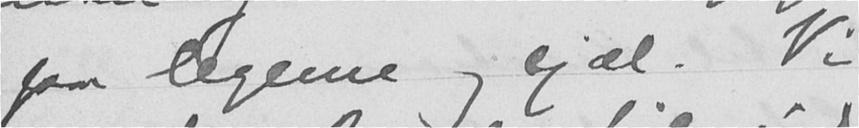paa legeme og sjæl. Vi
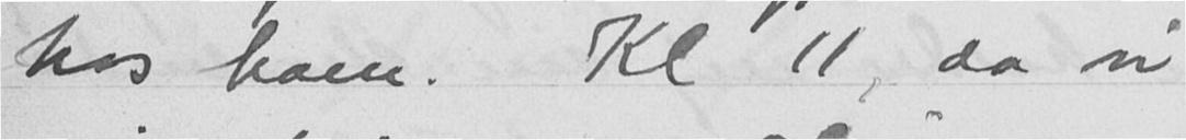hos ham. Kl. 11, da vi
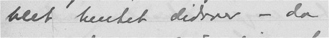blit hentet didover - da
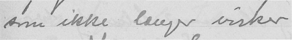som ikke længer virker
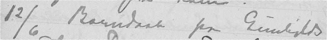12/6 Barnedaab for Gunhilds
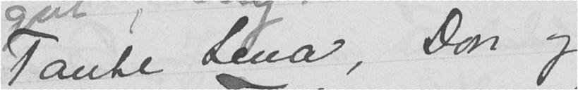Tante Lena, Don og
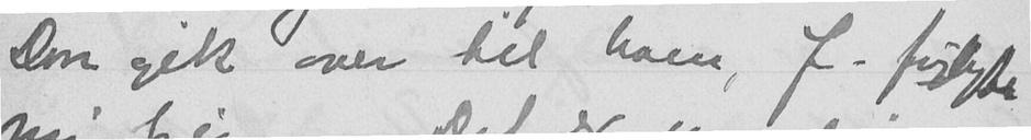Don gik over til ham, J. fulgte
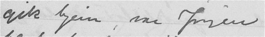gik hjem var Jørgen
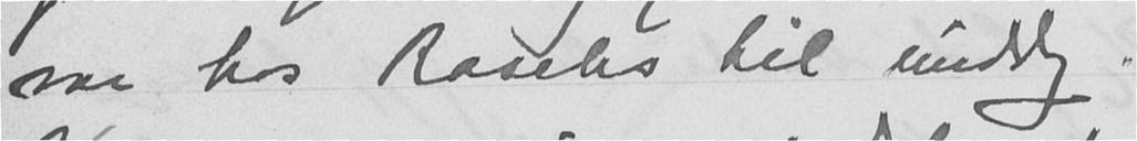var hos Raschs til middag.
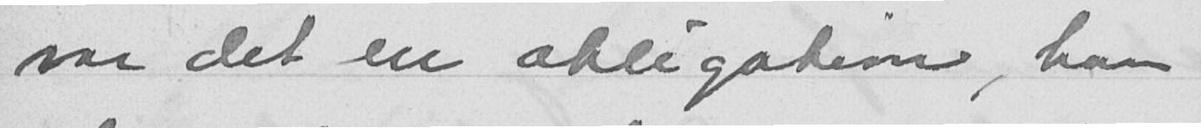var det en obligation, han
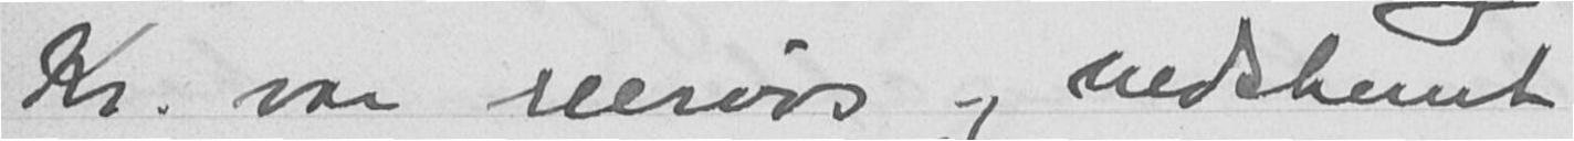Kr. var nervøs og nedstemt
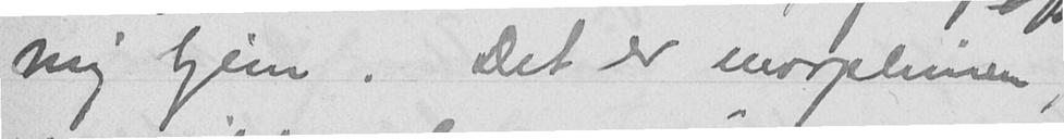mig hjem. Det er morphinen,
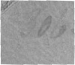306.
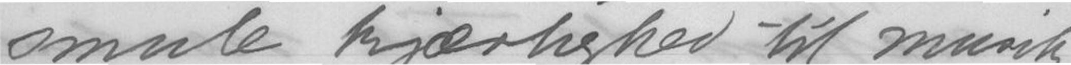smule kjærlighed - til musik
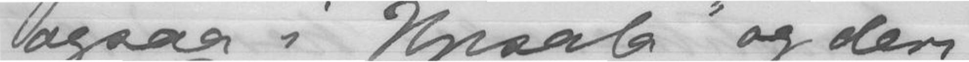ogsaa i Upsala" og deri
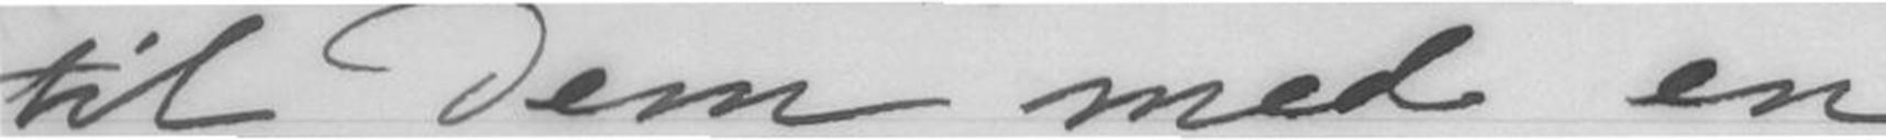til Dem med en
